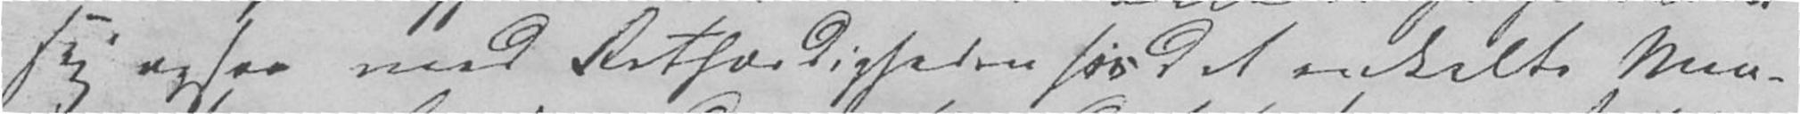sig ogsaa med Retfærdigheden hos det enkelte Men-
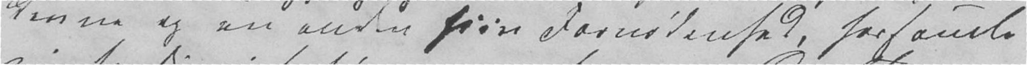denne og en anden hiin Fornødenhed, forsamle
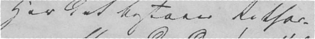Her det bestaaer Retfær-
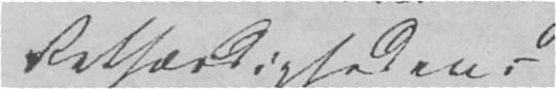Retfærdighedens
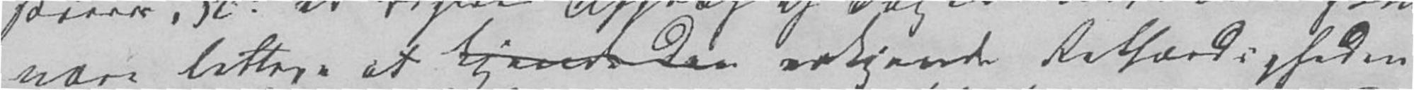være lettere at kjende den erkjende Retfærdigheden
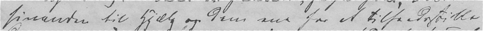hinanden til Hjælp og den ene for at tilfredsstille
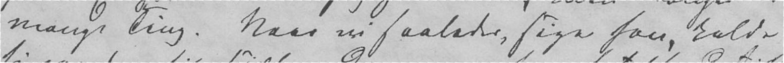mange Ting. Naar vi saaledes, siger han, kalde
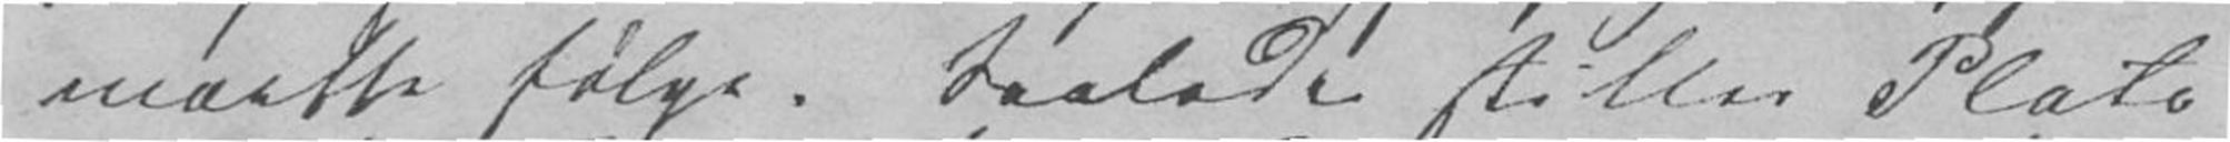maatte følge. Saaledes stiller Plato
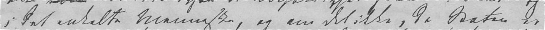i det enkelte Menneske, og om det ikke, da Staten er
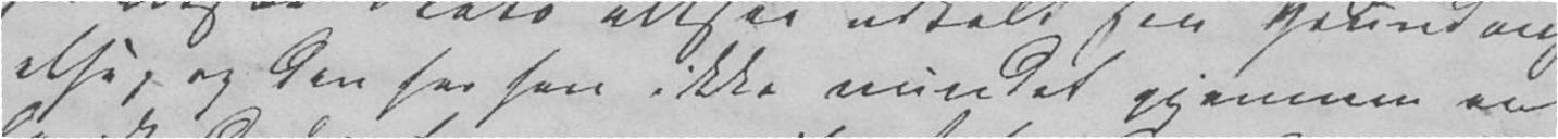else, og den har han ikke vundet gjennem en
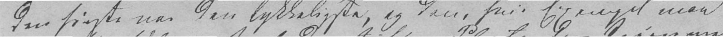den sidste var den lykkeligste, og den, hvis Exempel man
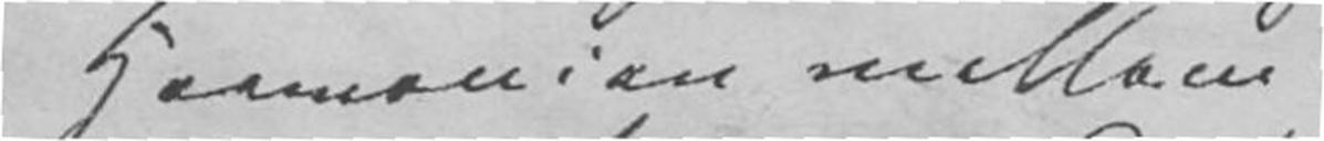Harmonien mellem
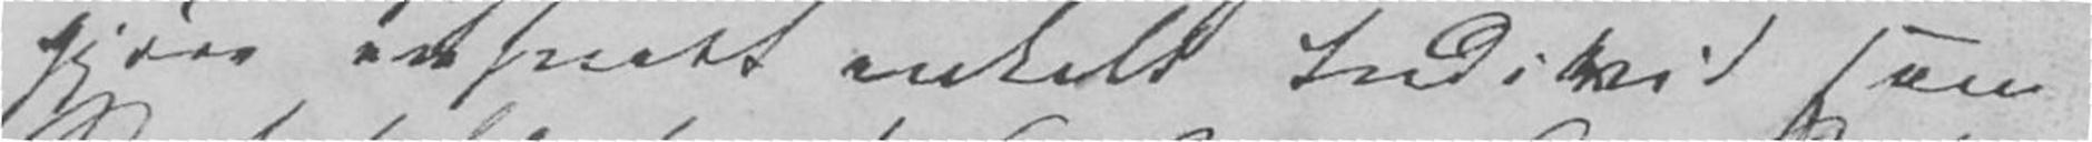gjøre ethvert enkelt Individ som
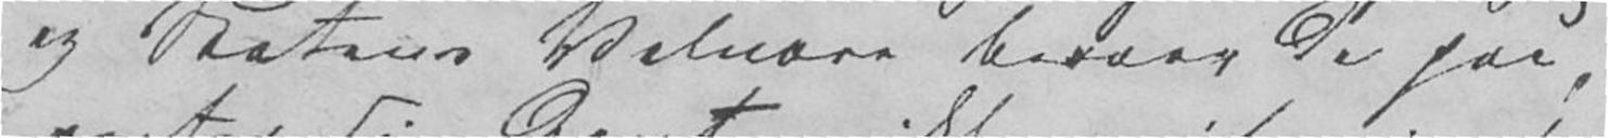og Statens Velvære beroer da paa
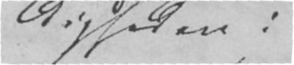digheden i
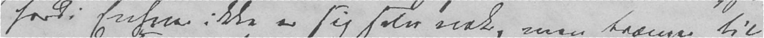fordi Enhver ikke er sig selv nok, men trænger til
